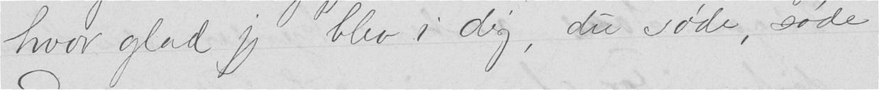hvor glad jeg blev i dig, du søde, søde
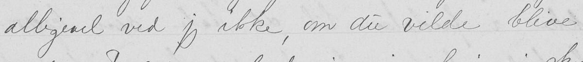alligevel ved jeg ikke, om du vilde blive
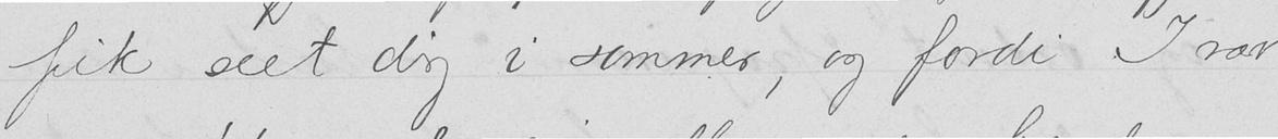fik seet dig i sommer, og fordi I var
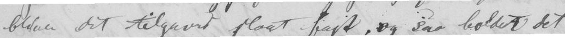bliver det tilgavns flot sagt, og saa holdet det
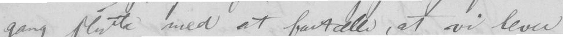gang slutte med at fortælle, at vi lever
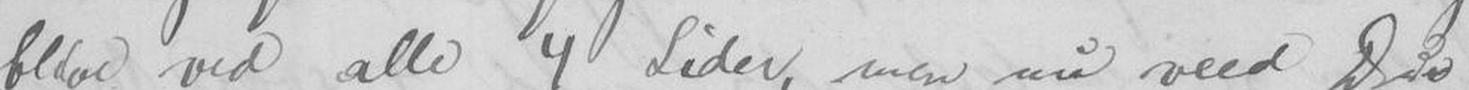blive ved alle 4 Sider, men nu veed Du
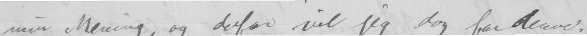min Mening, og derfor vil jeg dog for denne
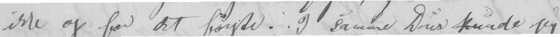ikke op for det første. I samme Dur kunde jeg
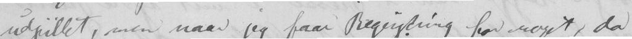udspillet, men naar jeg faar Begeistring for noget, da
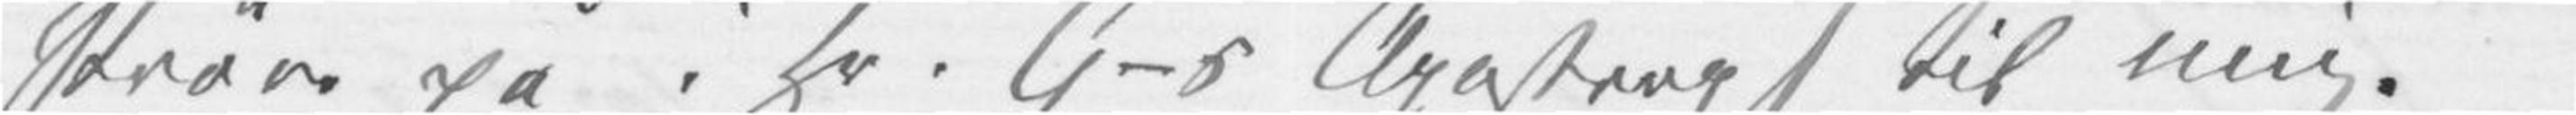Prøve på i Hr. G-s Apostroph til mig.
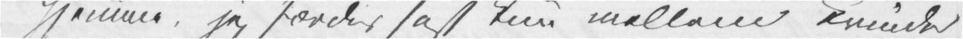hjemme, jeg færdes fast kun mellem Kvinder
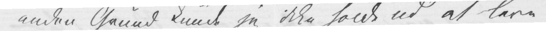anden Grund kunde jeg ikke holde ud at leve
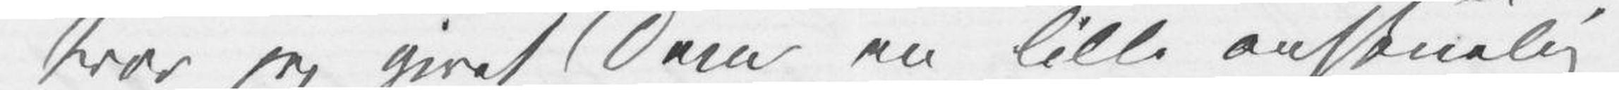tror jeg givet Dem en lille anskuelig
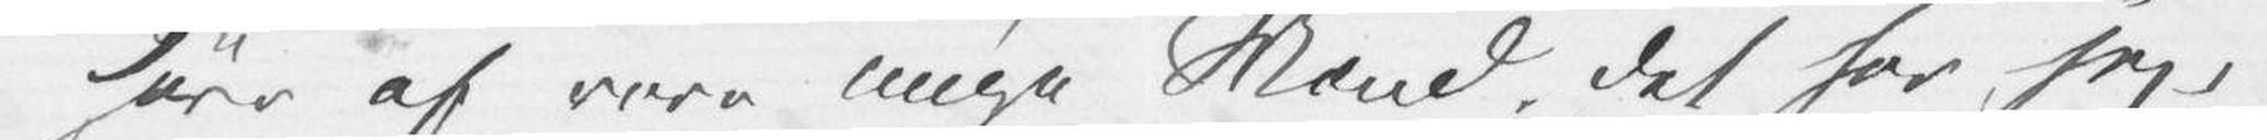høre af vore unge Mænd, det har jeg,
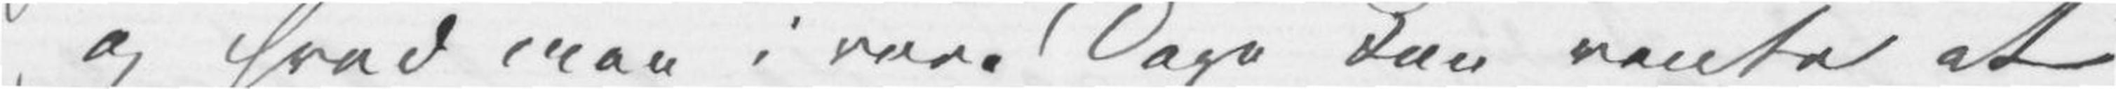og hvad man i vore Dage kan vente at
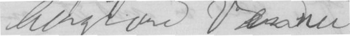hengivne Venner
